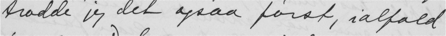trodde jeg det ogsaa først, ialfald
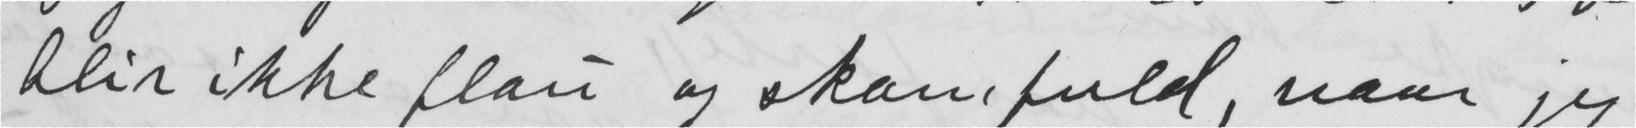blir ikke flau og skamfuld, naar jeg
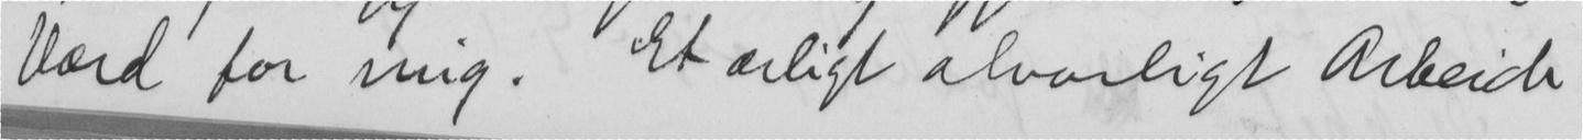Værd for mig. Et ærligt alvorligt Arbeide
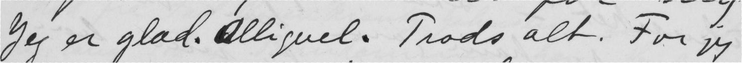Jeg er glad. Alligvel. Trods alt. For jeg
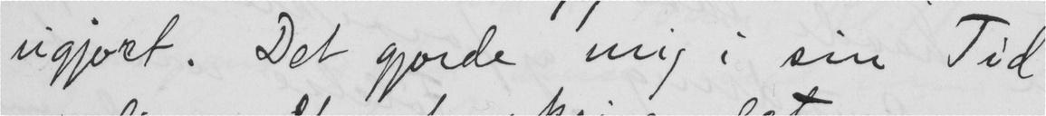ugjort. Det gjorde mig i sin Tid
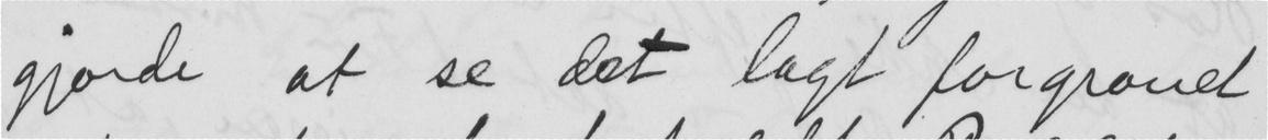gjorde at se det lagt forgrovet
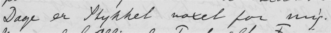Dage er Stykket voxet for mig.
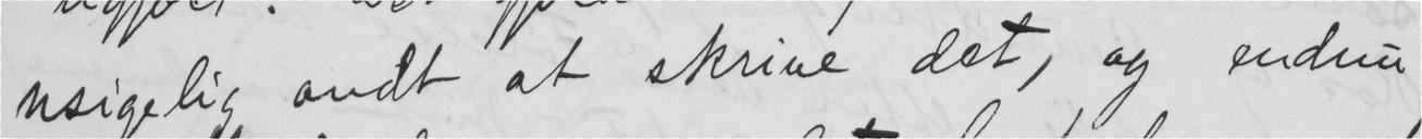usigelig ondt at skrive det, og endnu
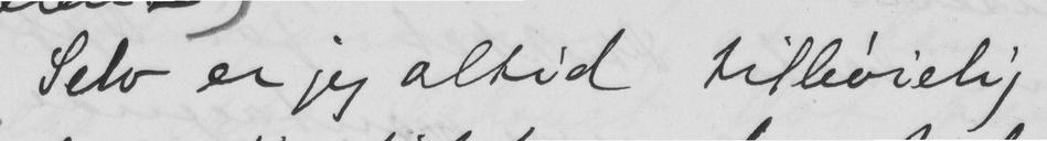Selv er jeg altid tilbøielig
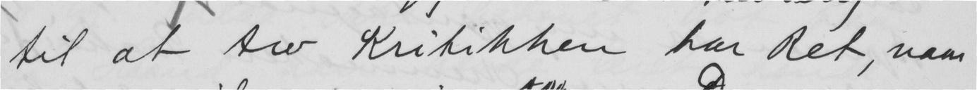til at tro Kritikken har Ret, naar
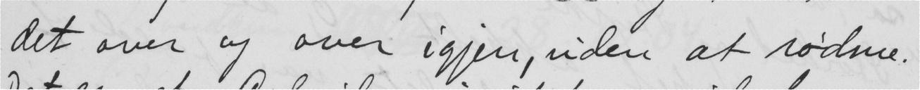det over og over igjen, uden at rødme.
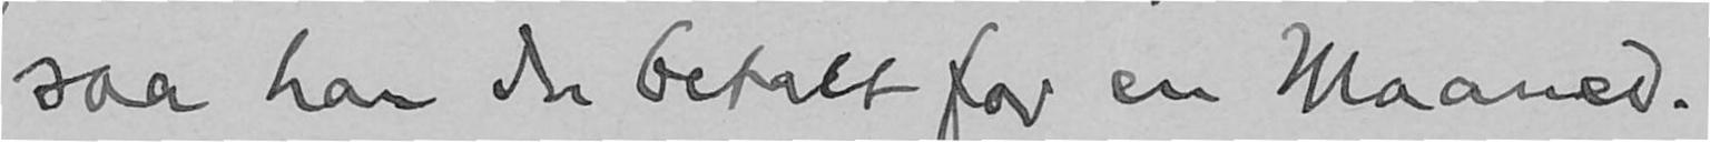saa har du betalt for en Maaned.
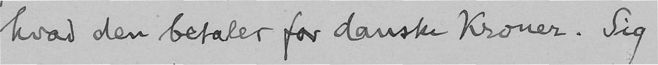hvad den betaler for danske Kroner. Sig
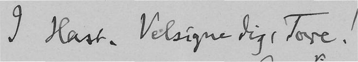I Hast. Velsigne dig, Tore!
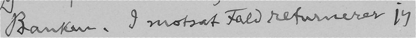Banken. I motsat Fald returnerer jeg
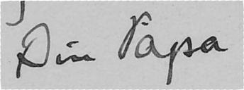Din Papa
